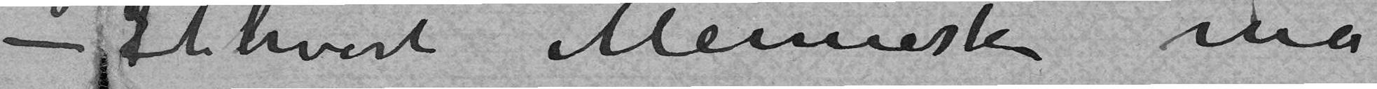– Ethvert Menneske må
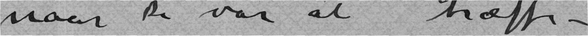naar De var at træffe –
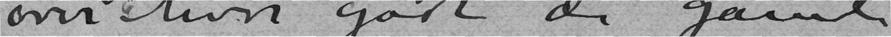over hvor godt de gamle
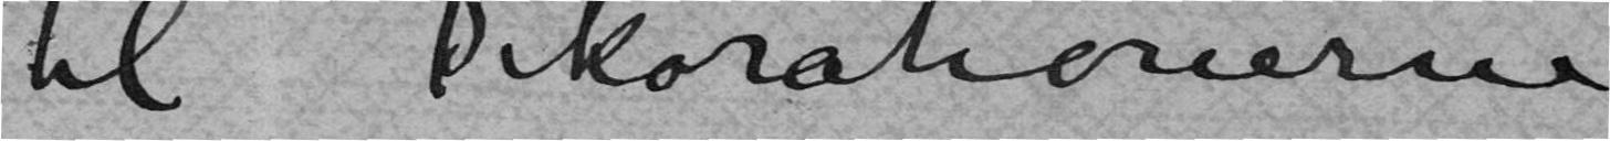til Dekorationerne
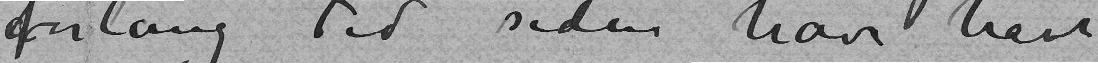for lang Tid siden have havt
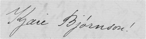Kjære Bjørnson!
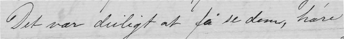Det var deiligt at få se dem, høre
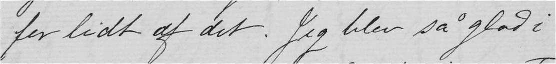for lidt af det. Jeg blev så glad i
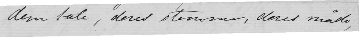dem tale, deres stemme, deret måde,
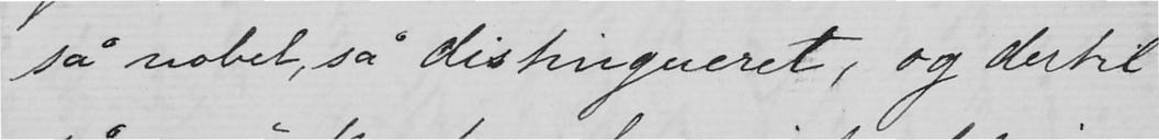så nobel, så distingveret, og dertil
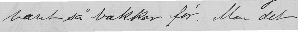været så vakker før. Men det
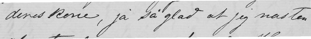deres kone, ja så glad at jeg næsten
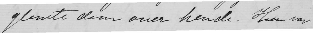glemte dem over hende. Hun var
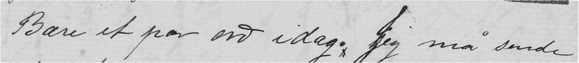Bare et par ord idag. Jeg må sende
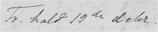Frg. hald 19de dcbr.
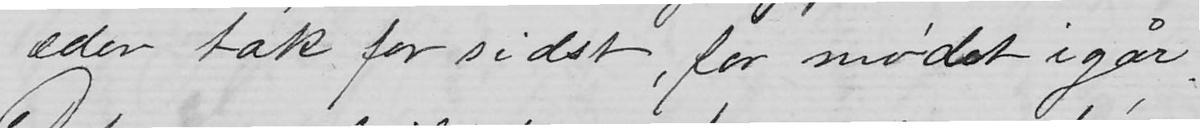eder tak for sidst, for mødet igår.
